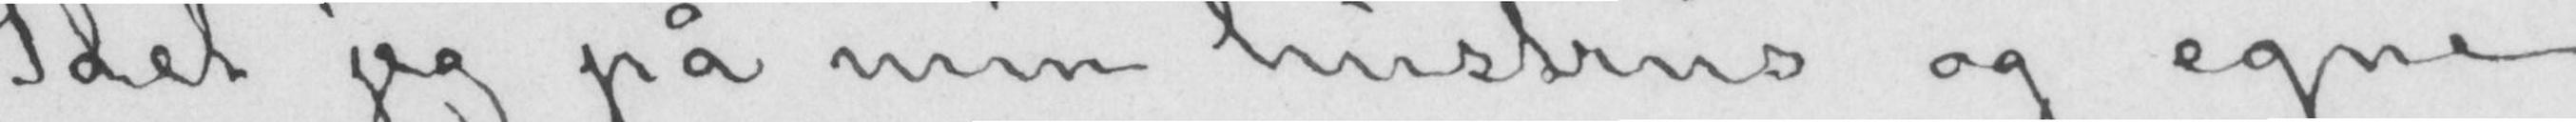Idet jeg på min hustrus og egne
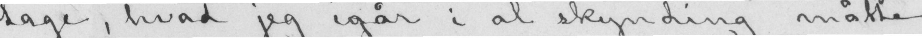tage, hvad jeg igår i al skynding måtte
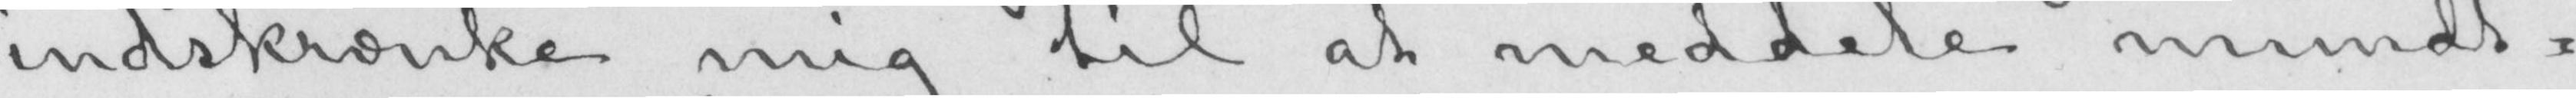indskrænke mig til at meddele mundt-
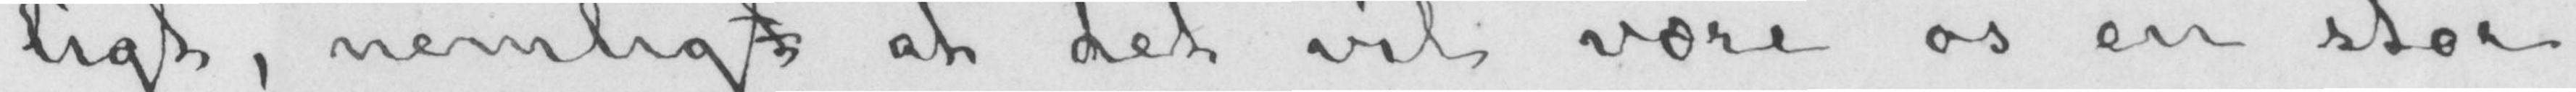ligt, nemligt at det vil være os en stor
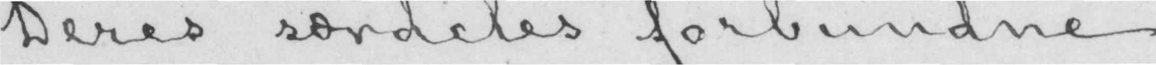Deres særdeles forbundne
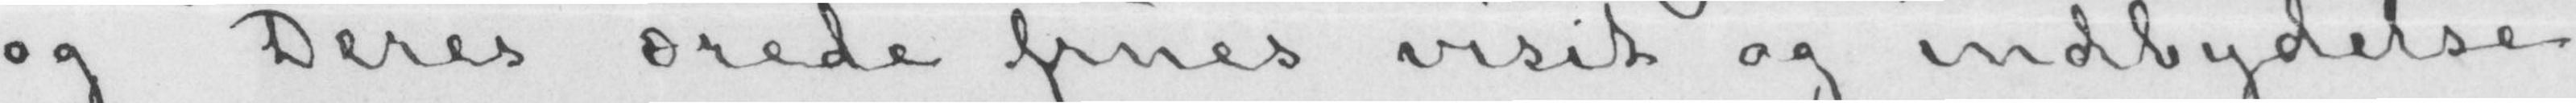og Deres ærede frues visit og indbydelse,
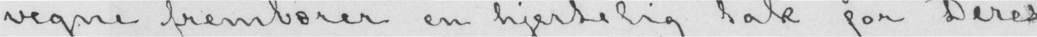vegne frembærer en hjertelig tak for Deres
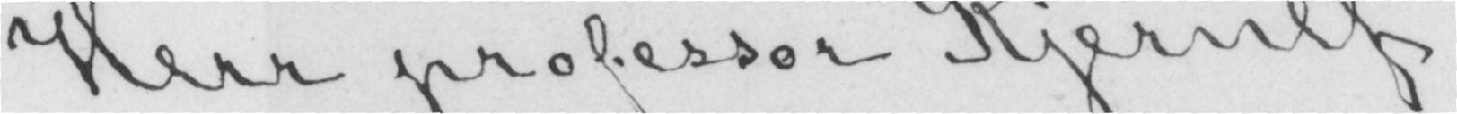Herr professor Kjerulf.
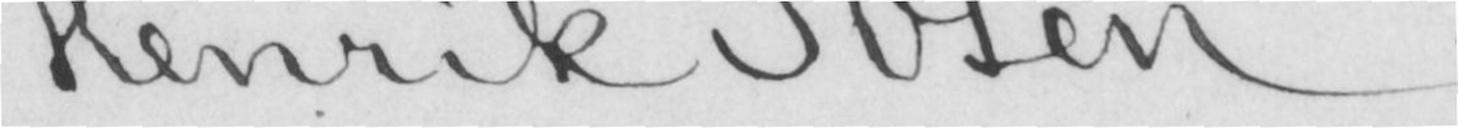Henrik Ibsen.
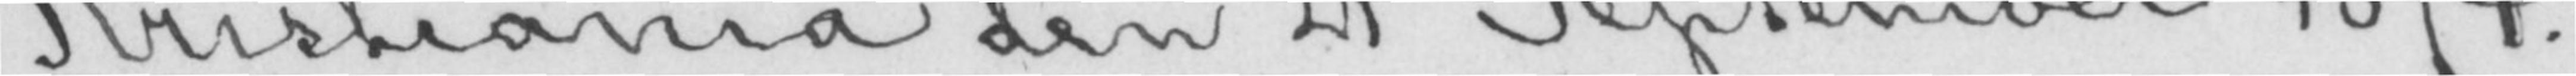Kristiania den 21de September 1874.
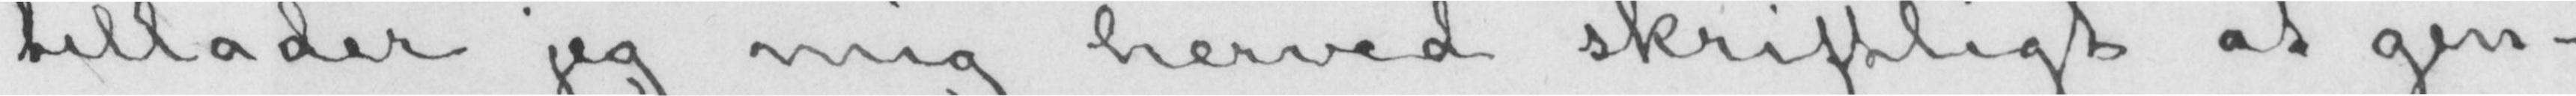tillader jeg mig herved skriftligt at gen-
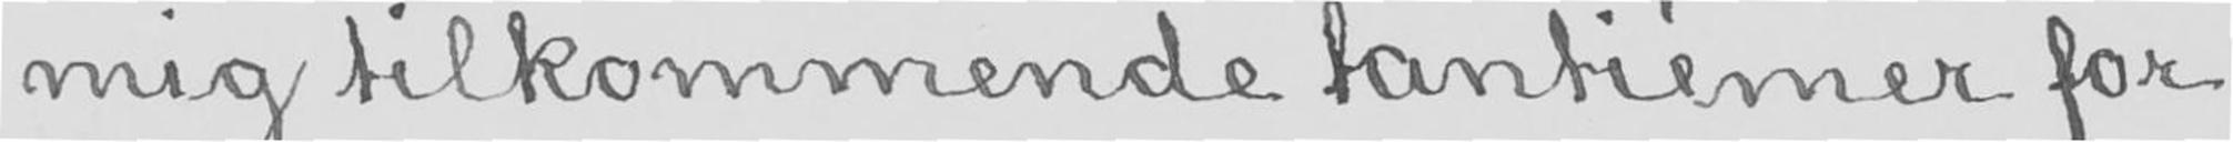mig tilkommende tantièmer for
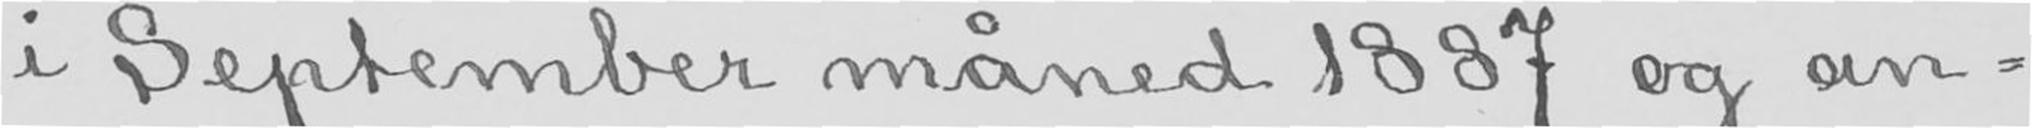i September måned 1887 og an-
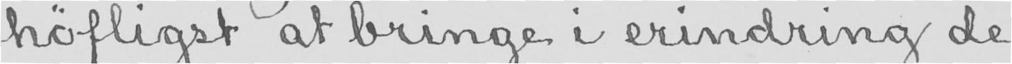høfligst at bringe i erindring de
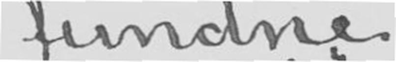fundne
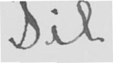Til
